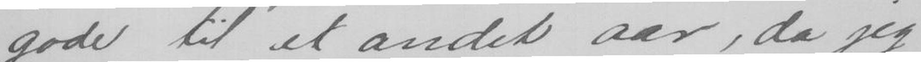gode til et andet aar, da jeg
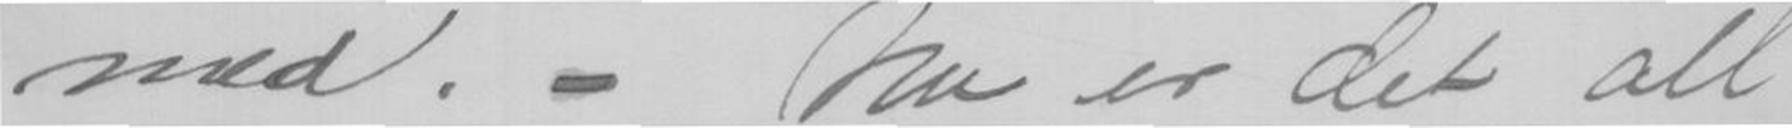med. - Nu er det all
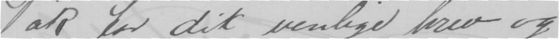Tak for dit venlige brev og
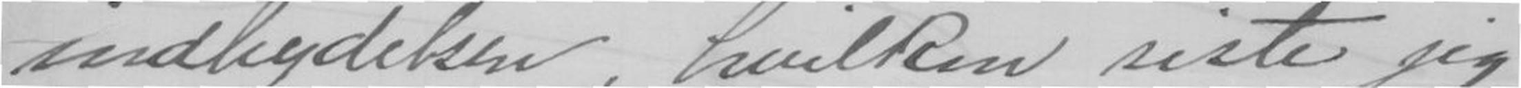indbydelsen, hvilket siste jeg
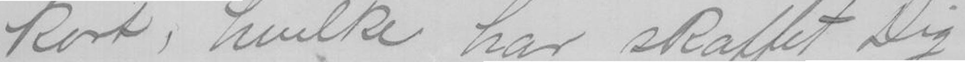kort, hvilke har skaffet Dig
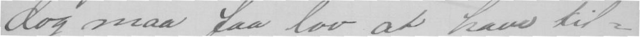dog maa faa lov at have til-
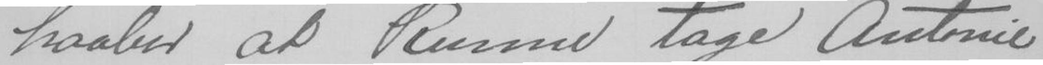haaber at kunne tage Antonie
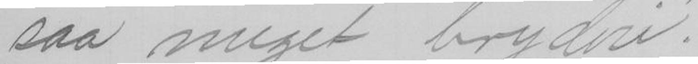saa meget bryderi.
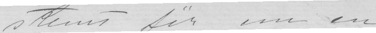stemt før om en
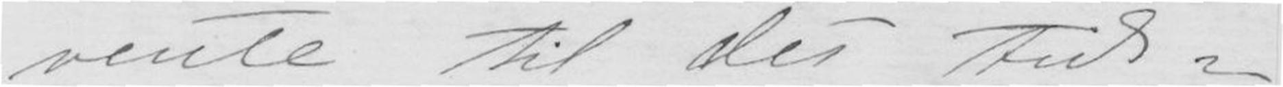vente til det tids-
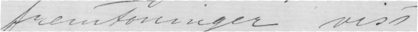fremtoninger vist
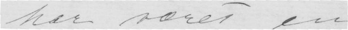har været en
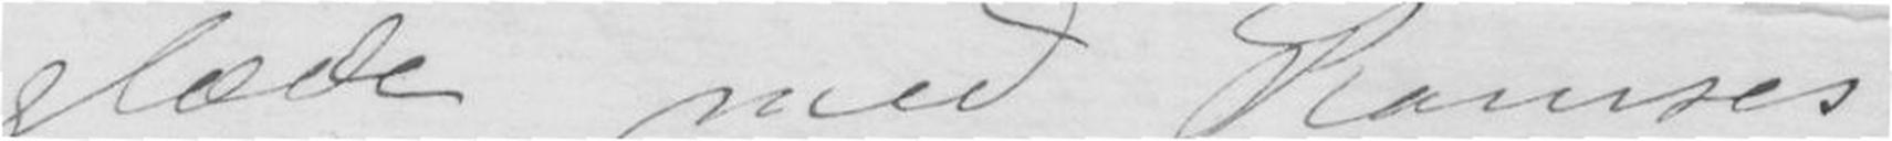glæde med Ramses
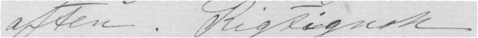aften. Rigtignok
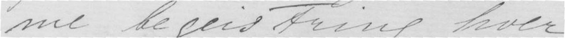me begeistring hver
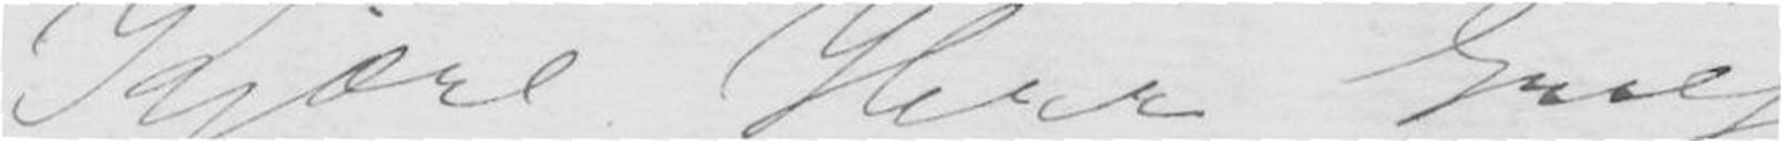Kjære Herr Grieg.
